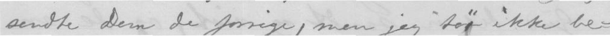sendte Dem de forrige, men jeg tør ikke be-
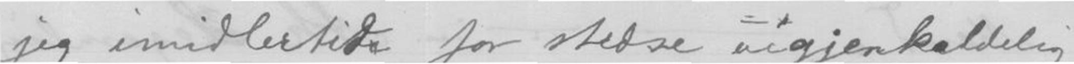jeg imidlertide for stedse uigjenkaldelig
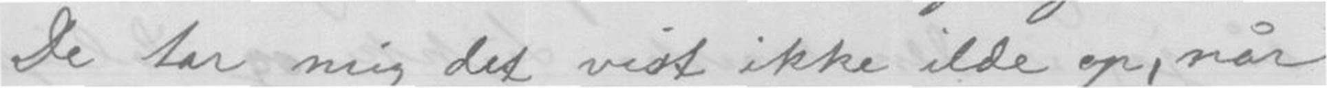De tar mig det vist ikke ilde op, når
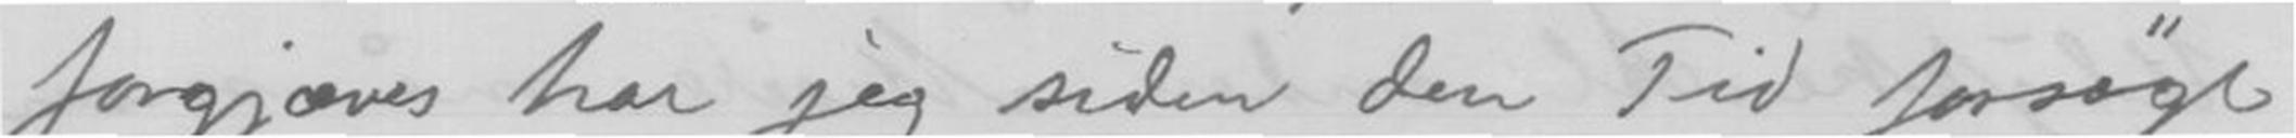forgjæves har jeg siden den Tid forsøgt
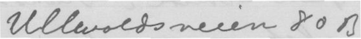Ullevoldsveien 80 B
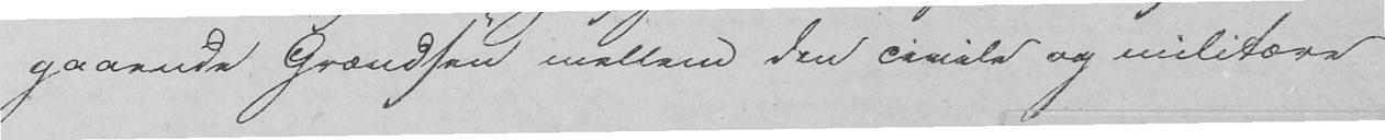gaaende Grændsen mellem den civile og militære
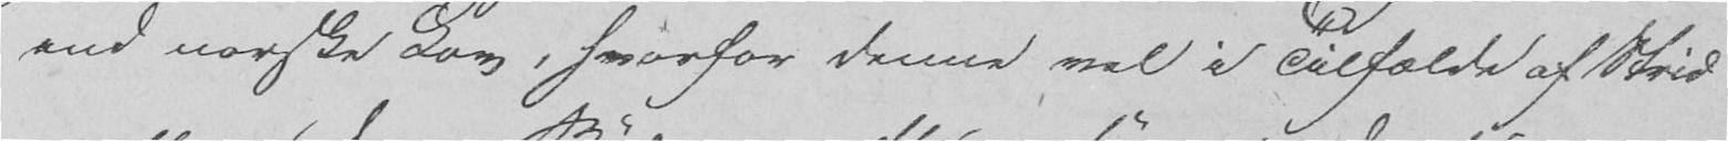end norske Lov, hvorfor denne vel i Tilfælde af Strid
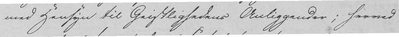med Hensyn til Geistlighedens Anliggender; herved
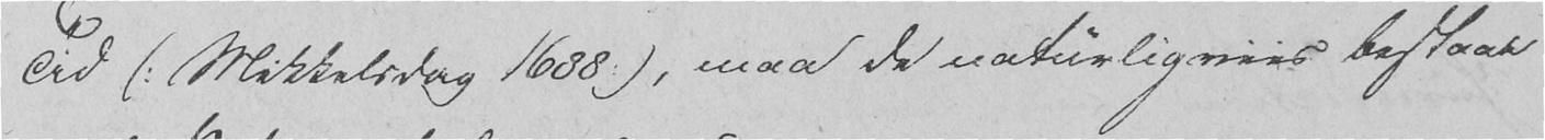Tid (: Mikkelsdag 1688 :), maa de naturligviis bestaae
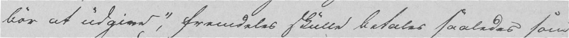bør at udgive", fremdeles skulle betales saaledes som
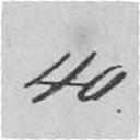40.
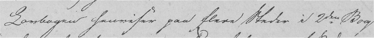Lovbogen henviser paa flere Steder i 2den Bog
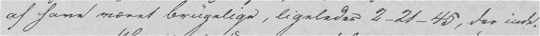af have været brugelige, ligeledes 2-21-45, der inde-
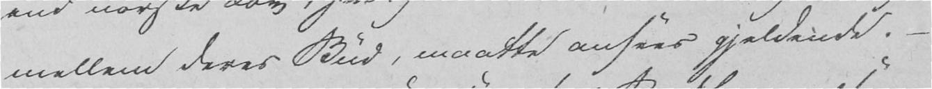mellem deres Bud, maatte ansees gjeldende. -
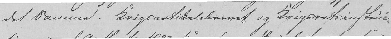det Samme. Krigsartikelsbrevet og Krigsretsinstruc-
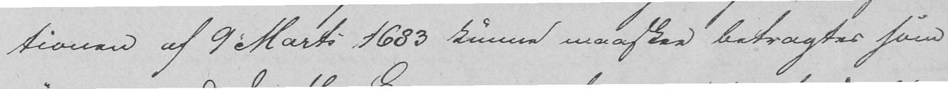tionen af 9 Marts 1683 kunne maaskee betragtes som
